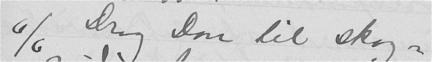6/6 Drog Don til skog-
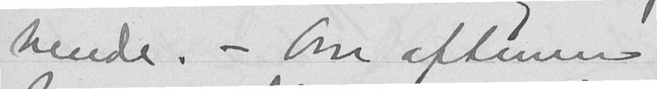hende. - Om aftenen
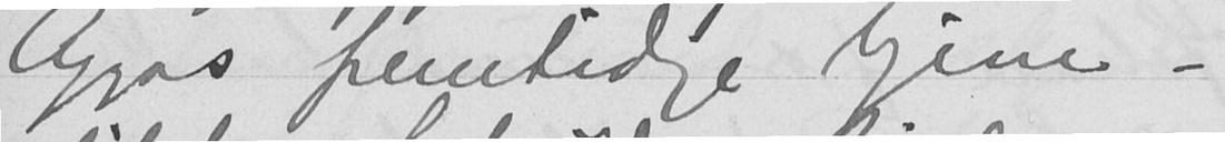Aggas fremtidige hjem -
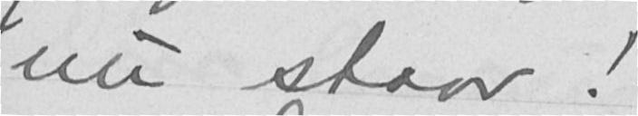nu staar!
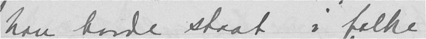han havde staat i folke
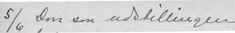5/6 Don saa udstillingen
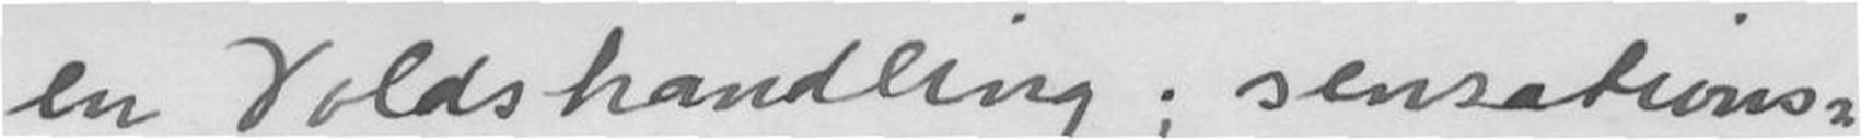en Voldshandling; sensations-
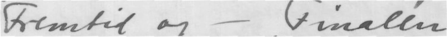Fremtid og - Finalen
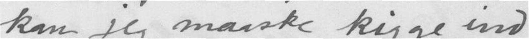kan jeg maaske kigge ind
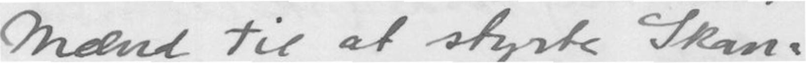Mænd til at styrte Skan-
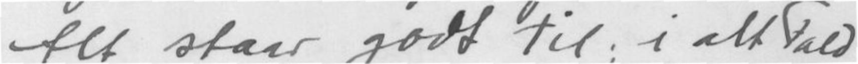Alt staar godt til; i alt Fald
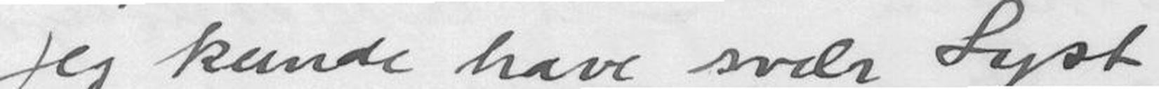Jeg kunde have svær Lyst
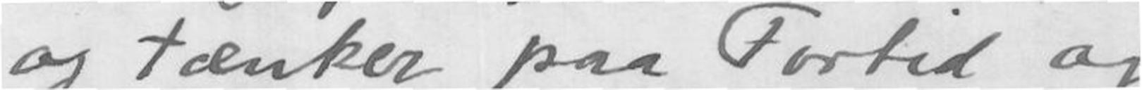og tænker paa Fortid og
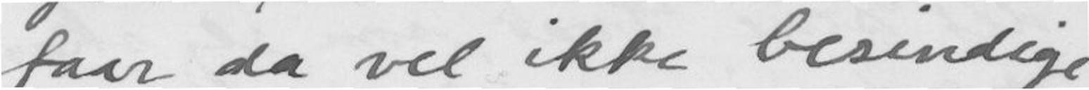faar da vel ikke besindige
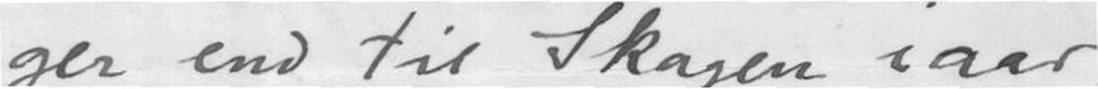ger end til Skagen iaar,
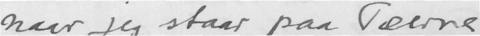naar jeg staar paa Tærne
